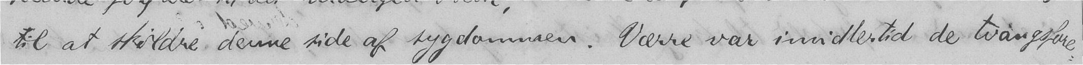til at skildre denne side af sygdommen. Værre var imidlertid de tvangsfore-
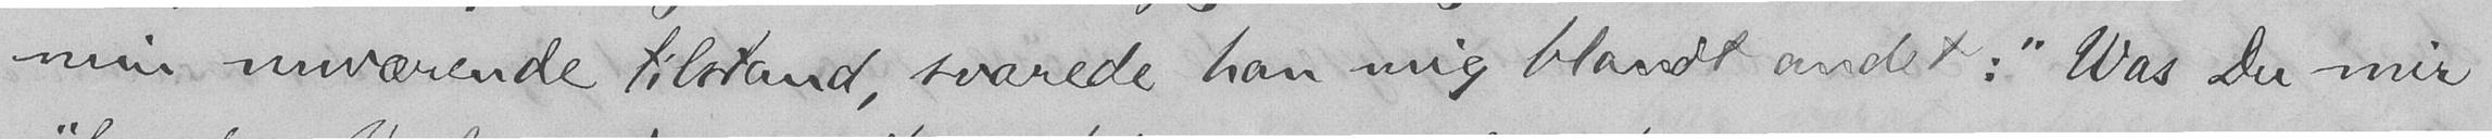min nuværende tilstand, svarede han mig blandt andet: "Was Du mir
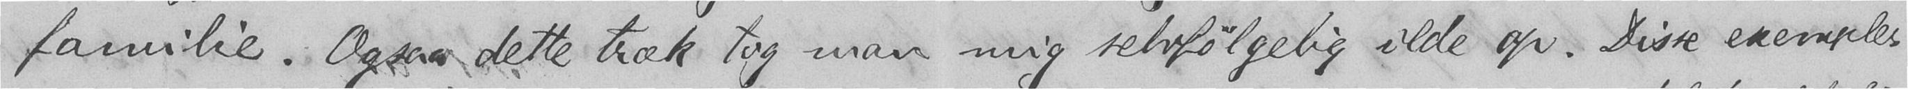familie. Ogsaa dette træk tog man mig selvfølgelig ilde op. Disse exempler
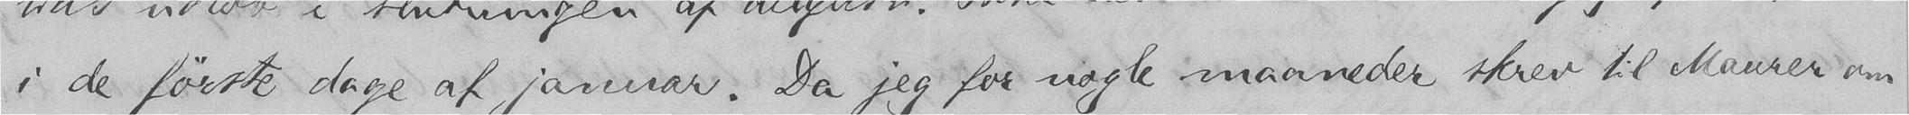i de første dage af januar. Da jeg for nogle maaneder skrev til Maurer om
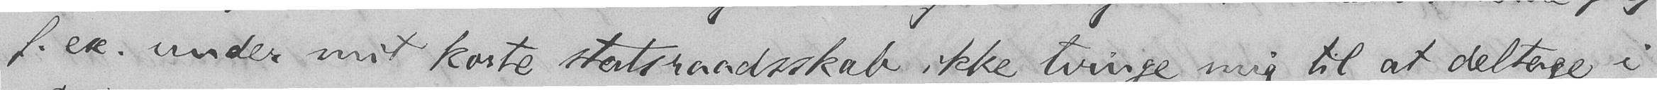f. ex. under mit korte statsraadsskab ikke tvinge mig til at deltage i
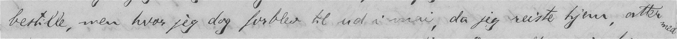bestille, men hvor jeg dog forblev til ud i mai, da jeg reiste hjem, atter med
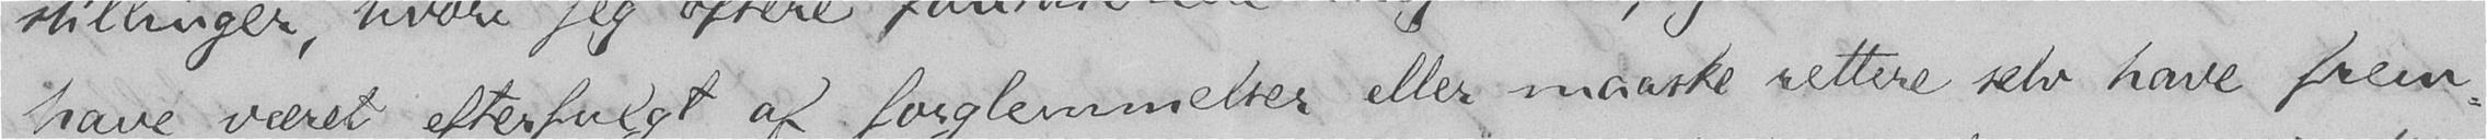have været efterfulgt af forglemmelser eller maaske rettere selv have frem-
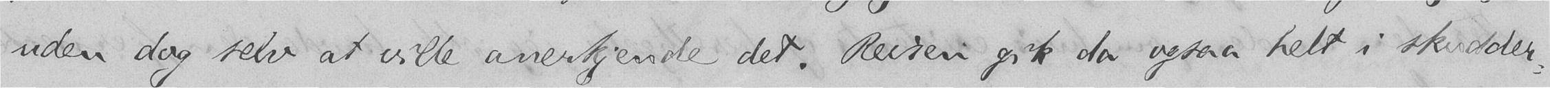uden dog selv at ville anerkjende det. Reisen gik da ogsaa helt i skudder-
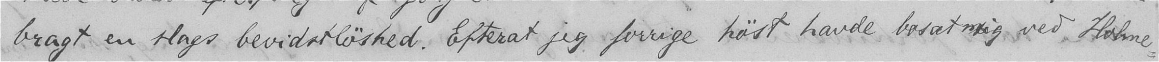bragt en slags bevidstløshed. Efterat jeg forrige høst havde bosat mig ved Holme-
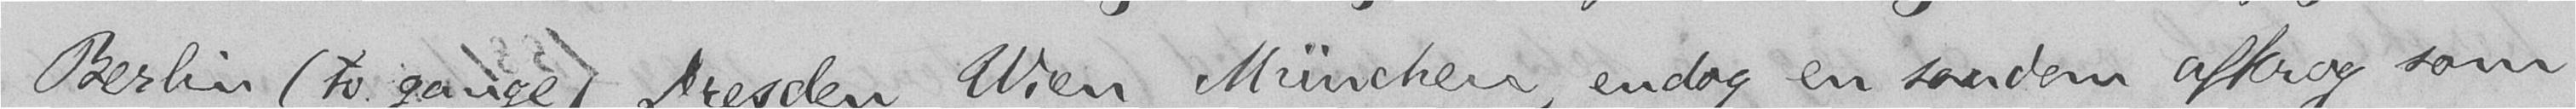Berlin (to gange), Dresden, Wien, München, endog en saaden afkrog som
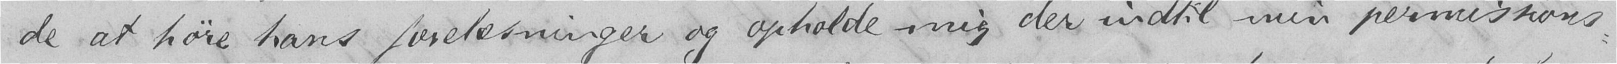de at høre hans forelæsninger og opholde mig der indtil min permissions-
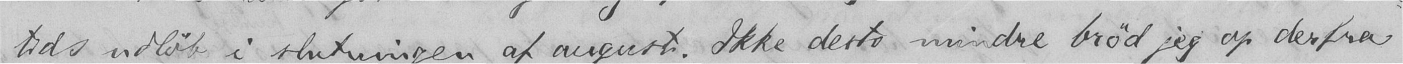tids udløb i slutningen af august. Ikke desto mindre brød jeg op derfra
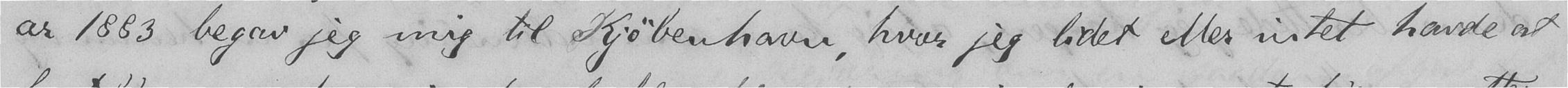ar 1883 begav jeg mig til Kjøbenhavn, hvor jeg lidet eller intet havde at
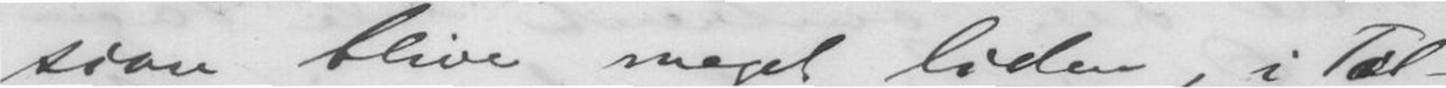sion blive meget liden, i Til-
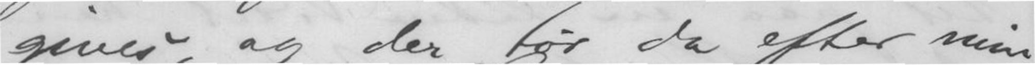gives, og der tør da efter min
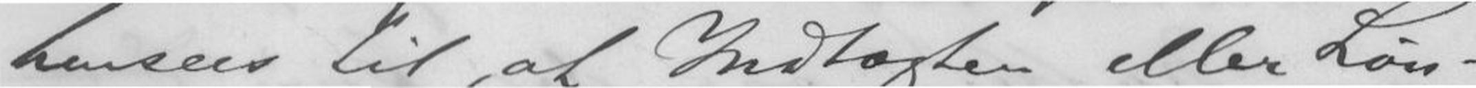hensees til, at Indtægten eller Løn-
